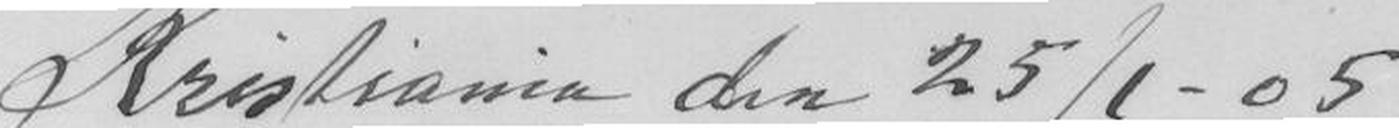Kristiania den 25/1-05
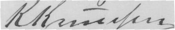K. Knutsen
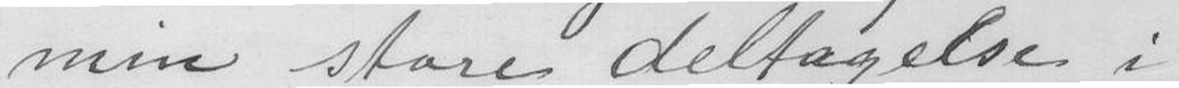min store deltagelse i
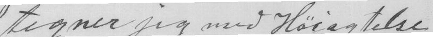tegner jeg med Høiagtelse
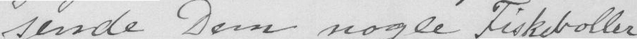sende Dem nogle Fiskeboller
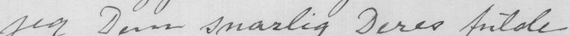jeg Dem snarlig Deres fulde
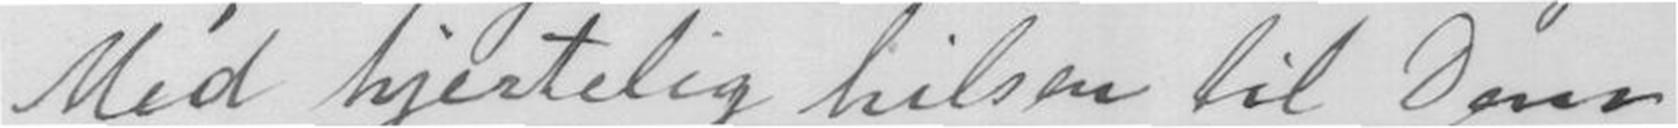Med hjertelig hilsen til Dem
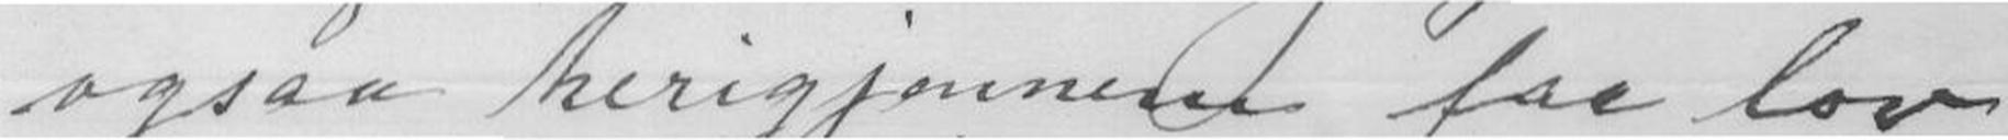ogsaa herigjennem faa lov
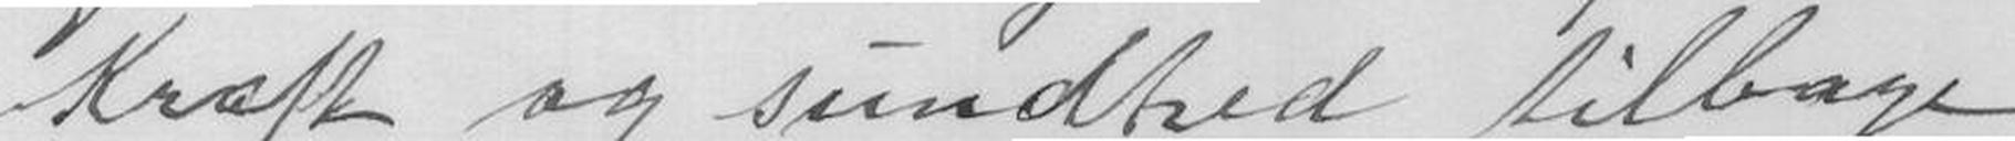Kraft og sundhed tilbage.
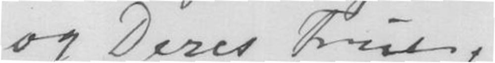og Deres Frue.
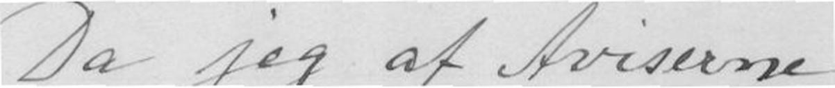Da jeg af Aviserne
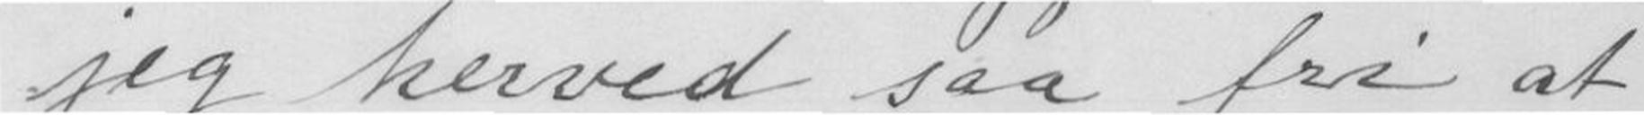jeg herved saa fri at
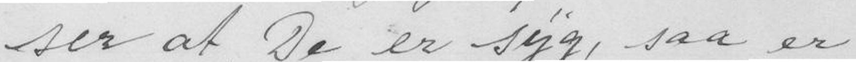ser at De er syg, saa er
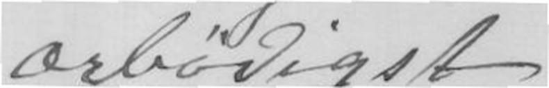ærgødigst
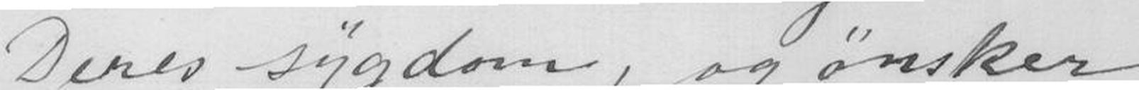Deres sygdom, og ønsker
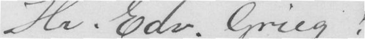Hr. Edv. Grieg !
